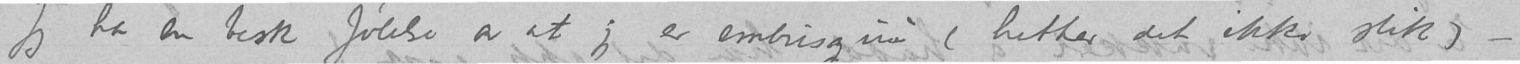Jeg har en besk følelse av at jeg er embusq nu (hetter det ikke slik) –
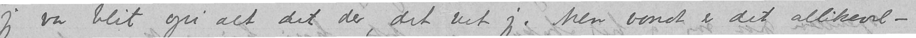jeg var blit opi alt det der, det vet jeg. Men vondt er det allikevel –
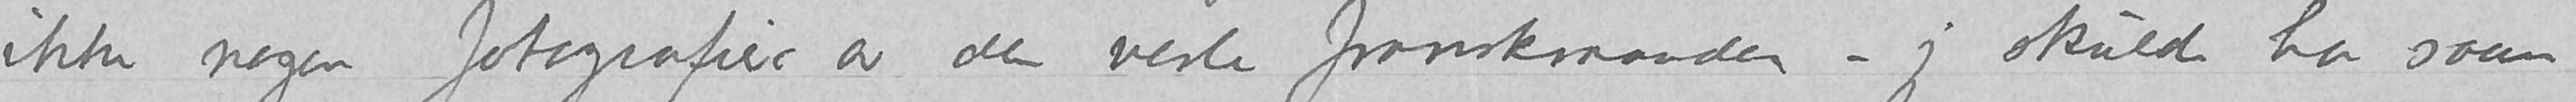ikke nogen fotografier av den vesle franskmanden – jeg skulde ha saan
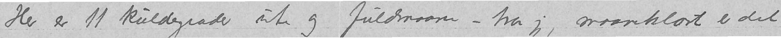Her er 11 kuldegrader ute og fuldmaane – tror jeg, maaneklart er det
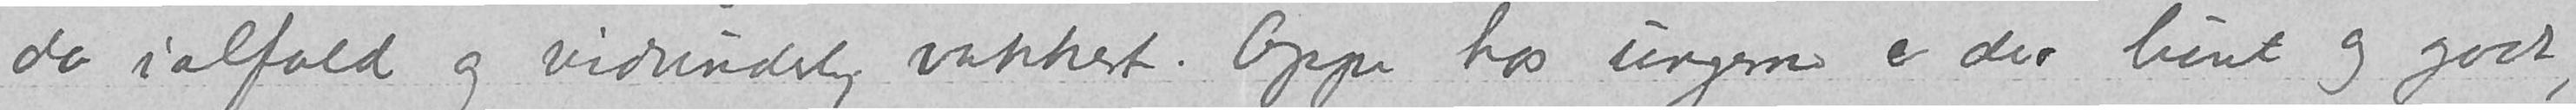da ialfald og vidunderlig vakkert. Oppe hos ungerne er der lunt og godt,
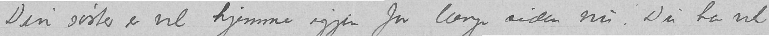Din søster er vel hjemme igjen for længe siden nu. Du har vel
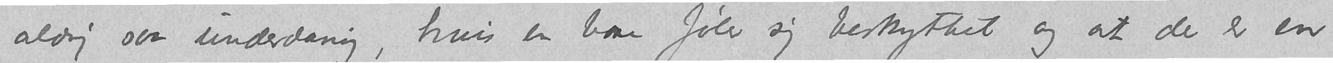aldrig saa underdanig, hvis en bare føler sig beskyttet og at der er en
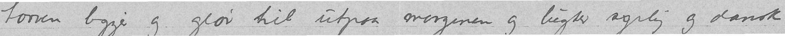torven ligger og glør til utpaa morgenen og lugter syrlig og dansk
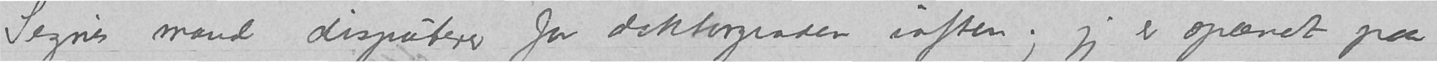Signes mand disputerer for doktorgraden i aften; jeg er spændt paa
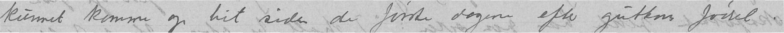kunnet komme op hit siden de første dagene efter guttens fødsel.
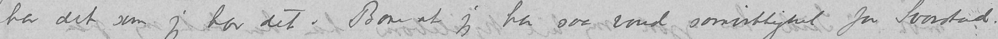har det som jeg har det. Bare at jeg har saa vond samvittighet for Svarstad.
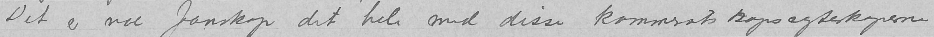Det er noe fanskap det hele med disse kammeratskapsægteskaperne
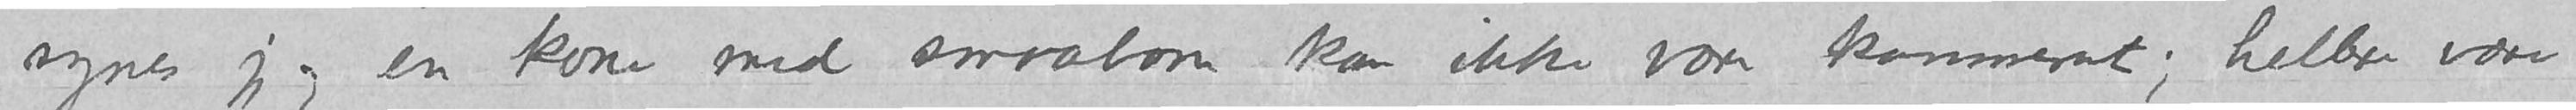synes jeg; en kone med smaabarn kan ikke være kammerat; hellere være
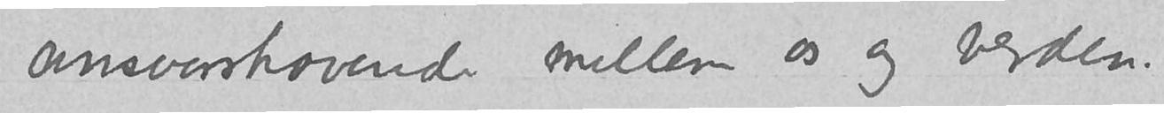ansvarshavende mellem os og verden.
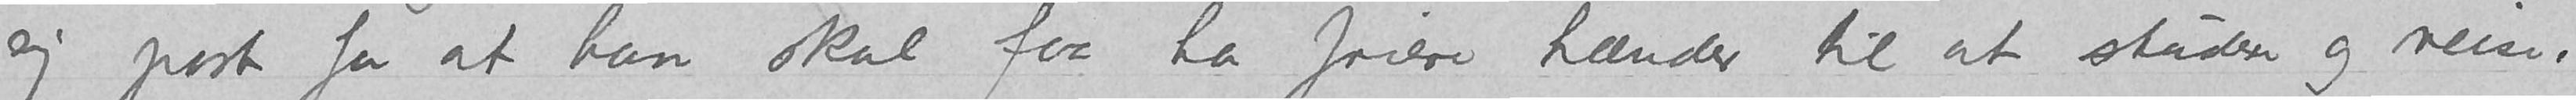sig post for at han skal faa ha friere hænder til at studere og reise.
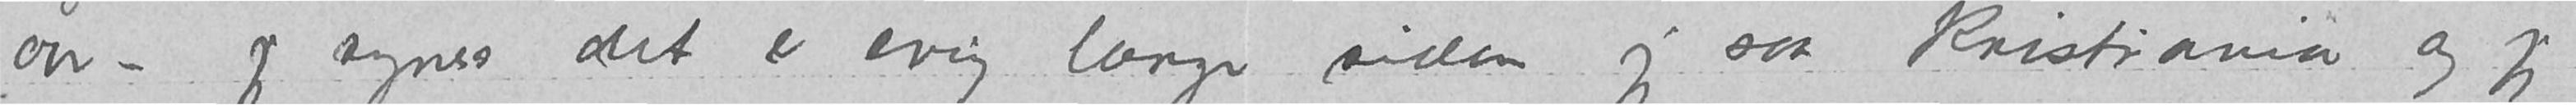aar – jeg synes det er evig længe siden jeg saa Kristiania og jeg
